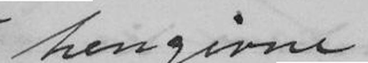hengivne
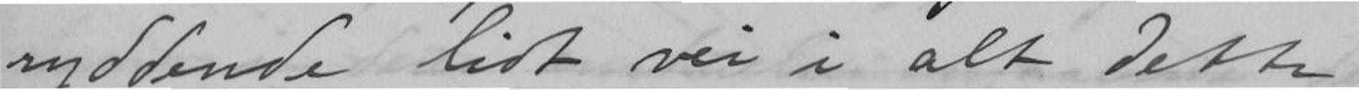ryddende lidt vei i alt dette
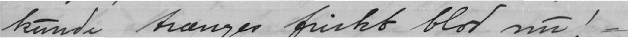kunde trænges friskt blod nu! -
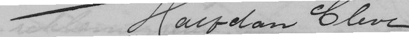Halvdan Cleve
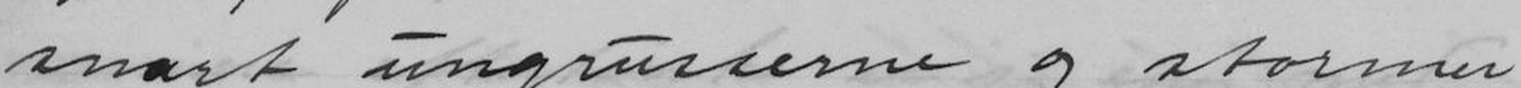snart ungrusserne og stormer
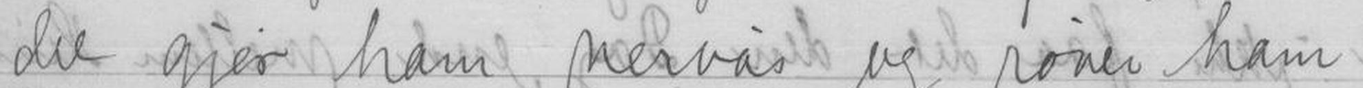det gjør ham nervøs og røver ham
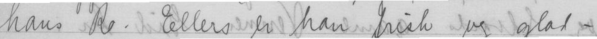hans Ro. Ellers er han frisk og glad -
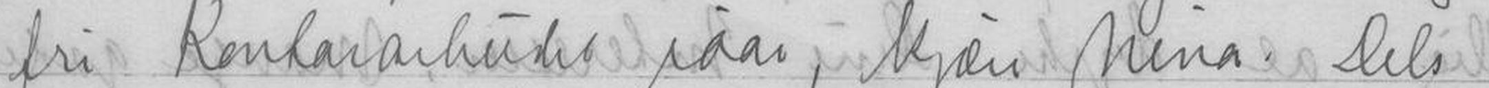fri Kontorarbeidet iaar, kjære Nina. Dels
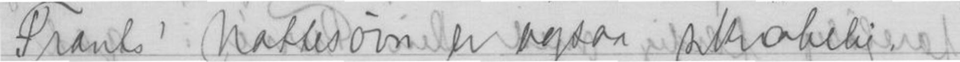Frants' Nattesøvn er ogsaa skrøbelig.
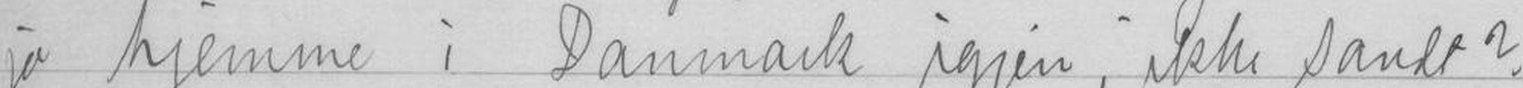jo hjemme i Danmark igjen, ikke sandt?
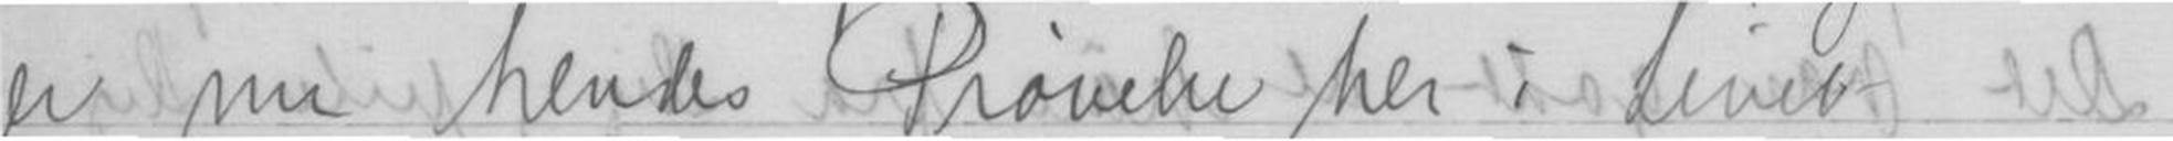er nu hendes Prøvelse her i Livet
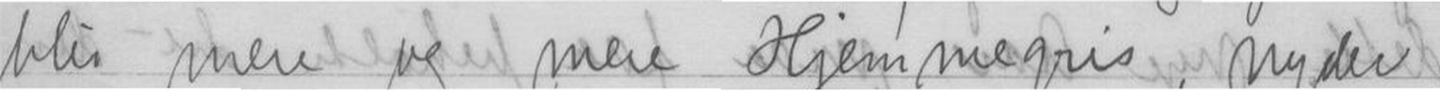blir mere og mere Hjemmegris, nyder
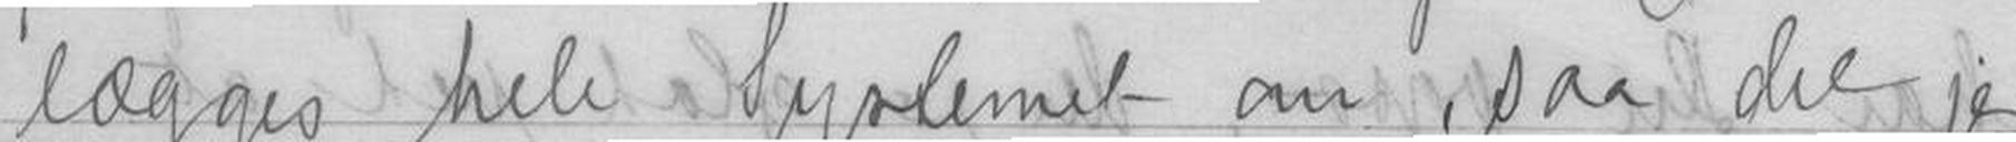lægges hele Systemet om, saa det jeg
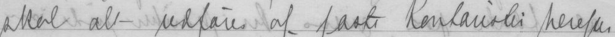skal alt udføres af faste Kontorister herefter.
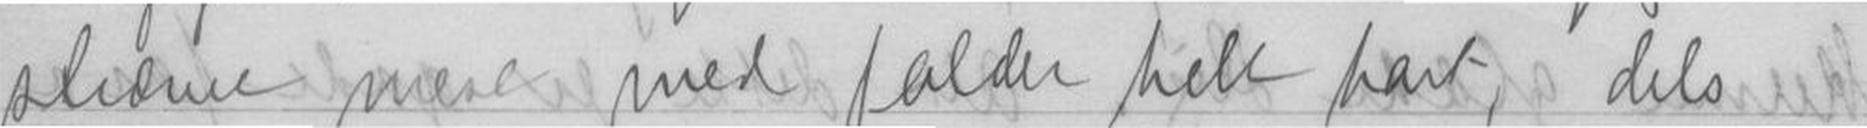strævet mest med falder helt bort, dels
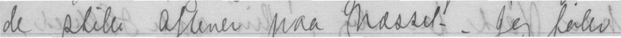de stille Aftener paa Næsset. Jeg føler
